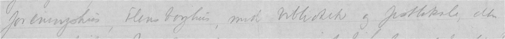foreningshus, Flensborghus, med bibliotek og festlokale, etter
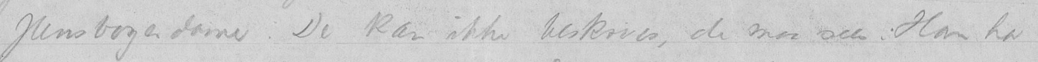flensborgerdame. De kan ikke beskrives, de maa sees. Han har
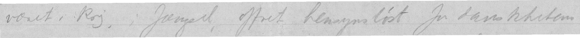været i krig, i fængsel, offret hensynsløst for danskhetens
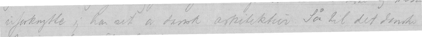uforknytte jeg har set av dansk arkitektur. Saa til det danske
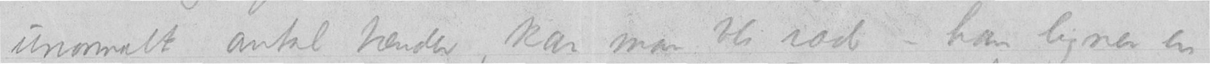unormalt antal tænder, kan man bli ræd – han ligner en
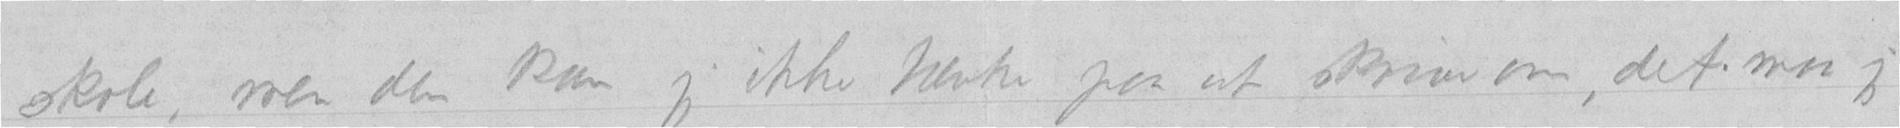skole, men den kan jeg ikke tænke paa at skrive om, det maa jeg
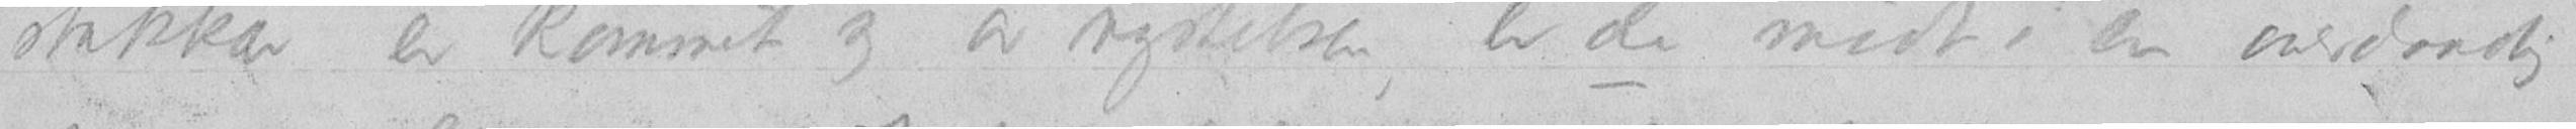stakkar er kommet sig av rystelsen, er de midt i en overdaadig
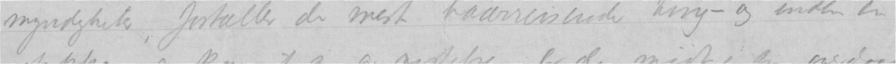myndigheter, fortæller de mest haarreisende ting – og inden en
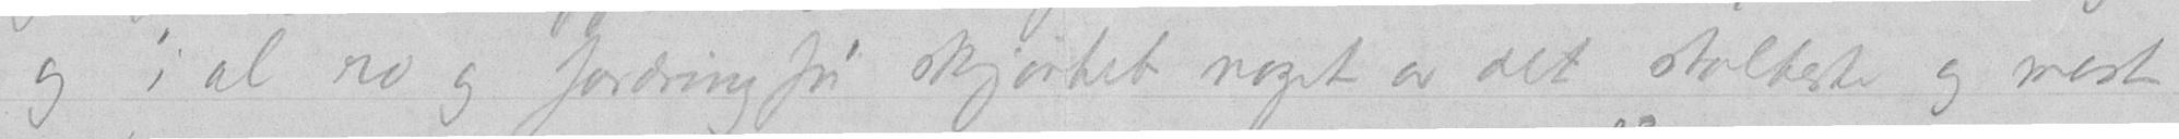og i al ro og fordringfri skjønhet noget av det stolteste og mest
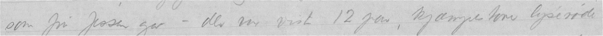som fru Jessen gav – der var vist 12 par, kjæmpestore lyserøde
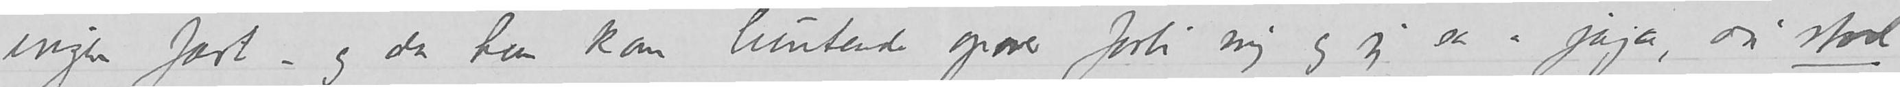ingen fart – og da han kom luntende opover forbi mig og jeg sa «jaja, du stod
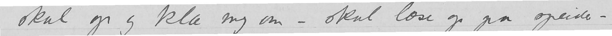Jeg skal op og klæ mig om - skal læse op paa speider-
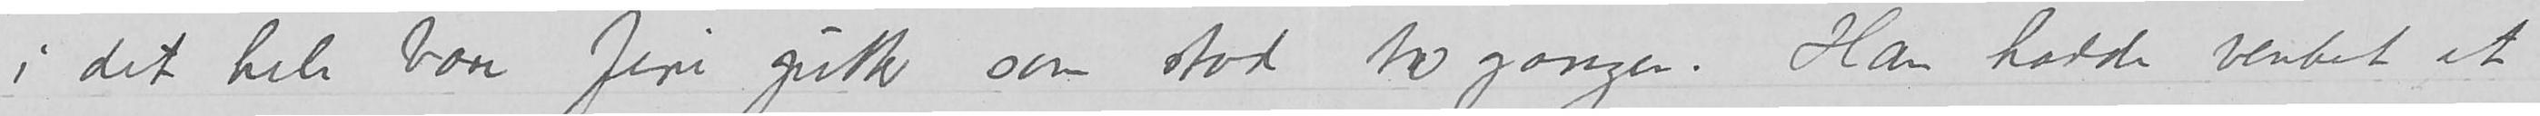i det hele bare fire gutter som stod to ganger. Han hadde ventet at
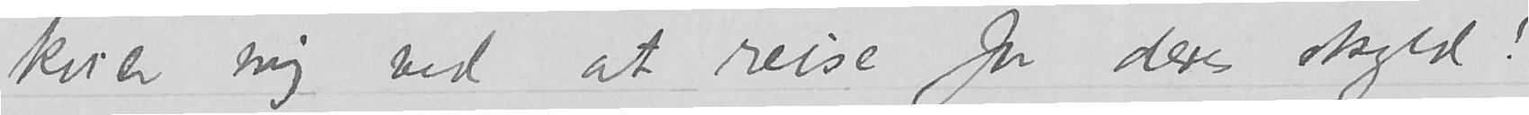kvier mig ved at reise for deres skyld!
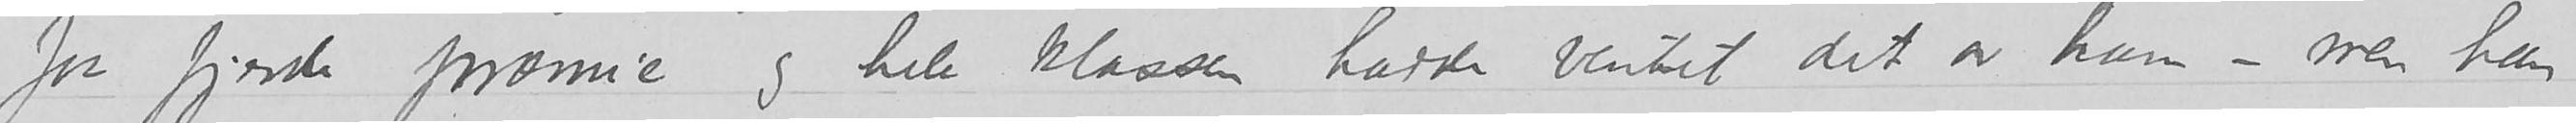faa fjerde præmie og hele klassen hadde ventet det av ham - men han
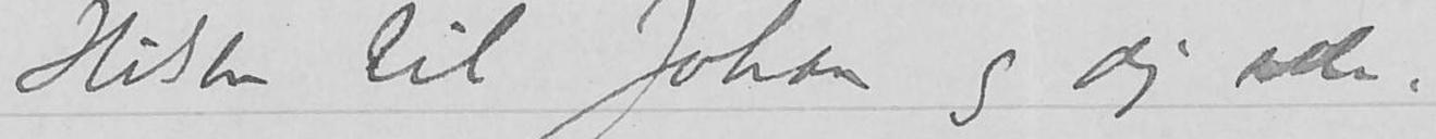Hilsen til Johan og dig selv.
